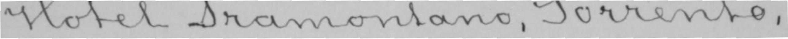Hotel Tramontano, Sorrento,
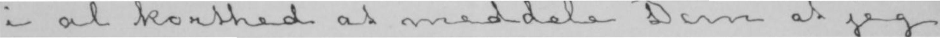i al korthed at meddele Dem at jeg
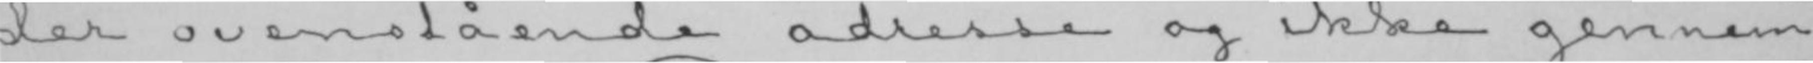der ovenstående adresse og ikke gennem
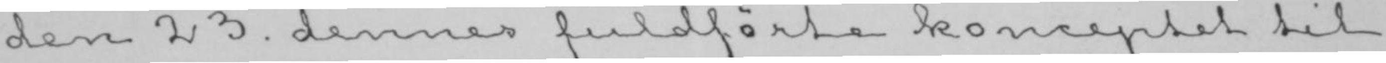den 23. dennes fuldførte konceptet til
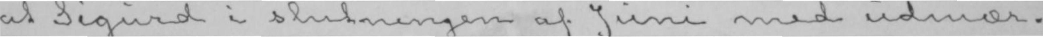at Sigurd i slutningen af Juni med udmær-
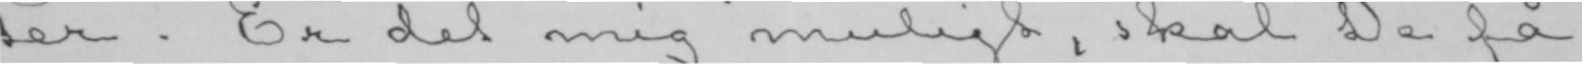ter. Er det mig muligt, skal De få
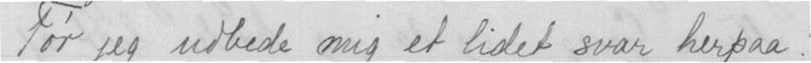Tør jeg udbede mig et lidet svar herpaa ?
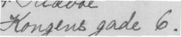Kongens gade 6.
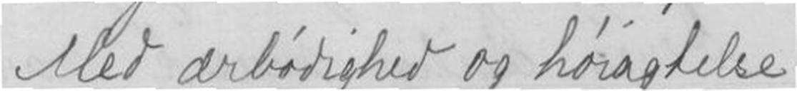Med ærbødighed og høiagtelse
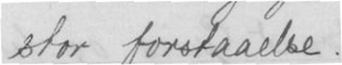stor forstaaelse.
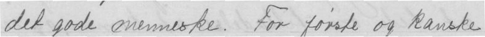det gode menneske. For første og kanske
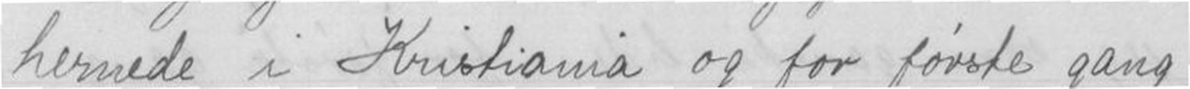hernede i Kristiania og for første gang
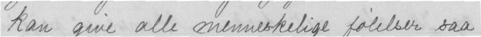kan give alle menneskelige følelser saa
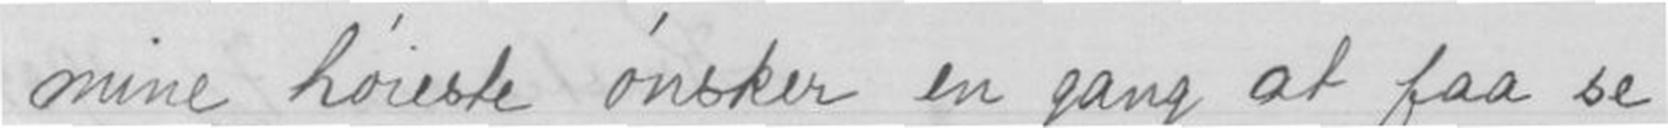mine høieste ønsker en gang at faa se
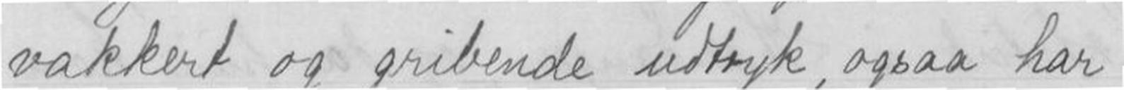vakkert og gribende udtryk, ogsaa har
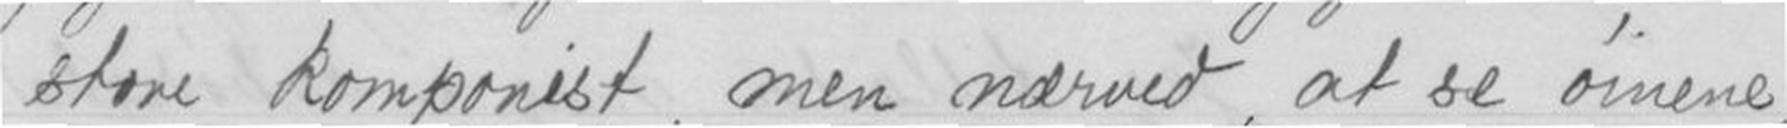store komponist, men nærved, at se øinene,
